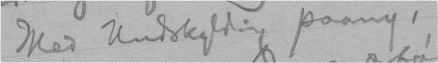Med Undskylding paany,
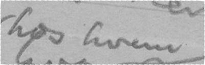hos hvem
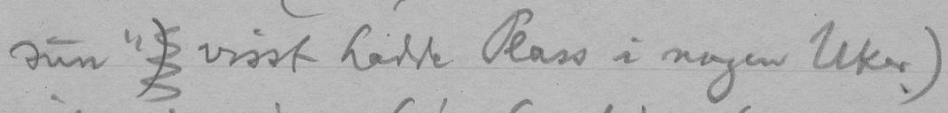sun") visst hadde Plass i nogen Uker)
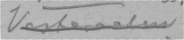Vesteraalen
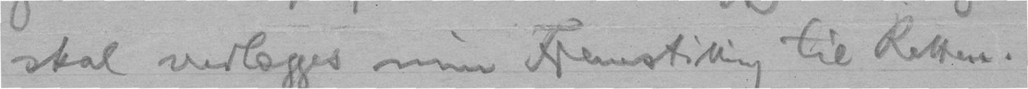skal vedlægges min Fremstilling til Retten.
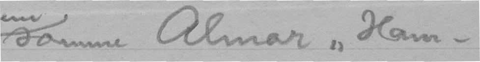samme Almar "Ham-
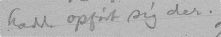hadde opført sig der.
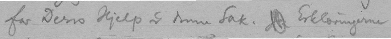for Deres Hjelp i denne Sak. Jeg Erklæringerne
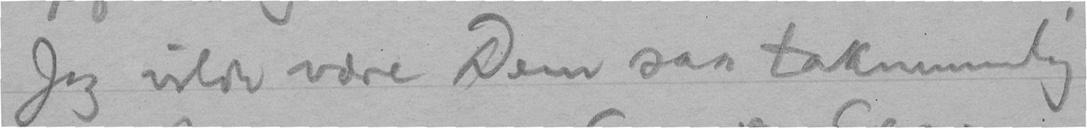Jeg vilde være Dem saa taknemmelig
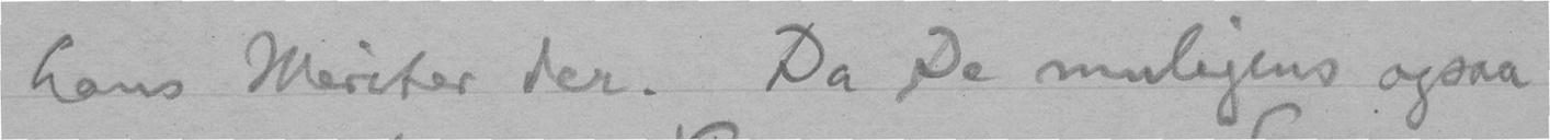hans Meriter der. Da De muligens ogsaa
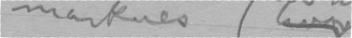marknes (hvor
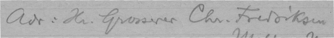Adr: Hr. Grosserer Chr. Fredriksen
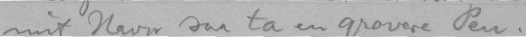mit Navn saa ta en grovere Pen.
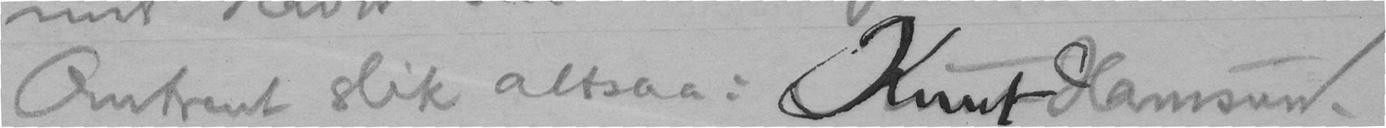Omtrent slik altsaa: Knut Hamsun.
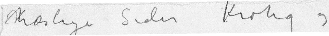hæslige Sider Krohg og
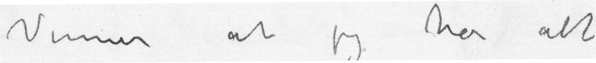Venner at jeg har alt
